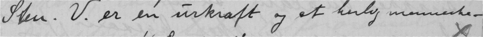Sten. V. er en urkraft og et herlig menneske-
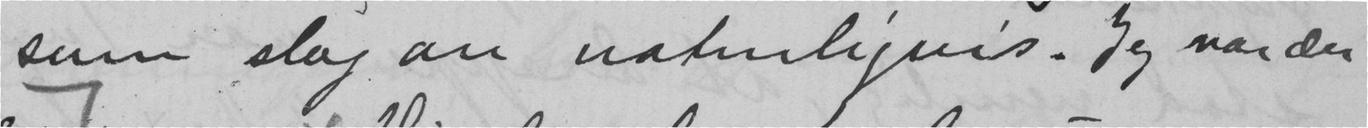som slog an naturligvis. Jeg var der
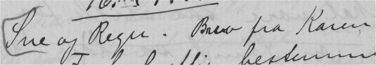Sne og Regn. Brev fra Karen
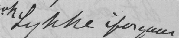Lykke iforgaar
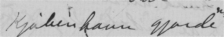Kjøbenhavn gjorde
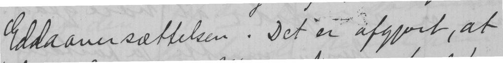Eddaoversættelsen. Det er afgjort, at
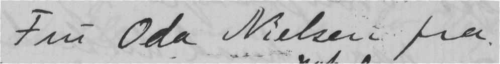Fru Oda Nielsen fra
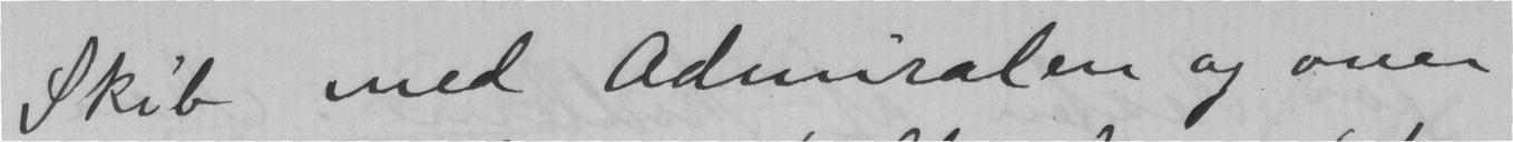Skib med Admiralen og over
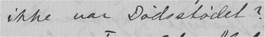ikke var Dødsstødet?
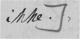ikke.
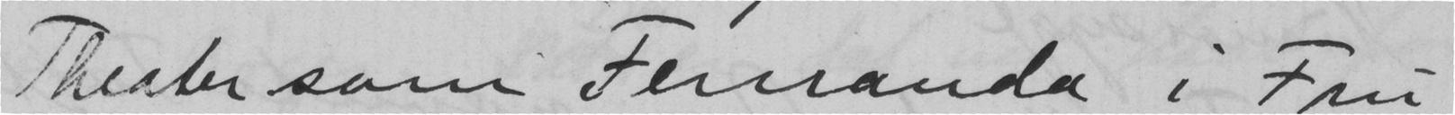Theater som Fernanda i Fru
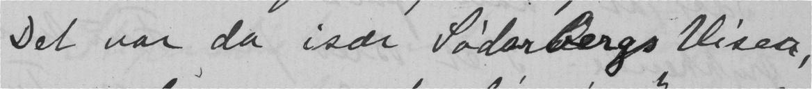Det var da især Sødarbergs Visen,
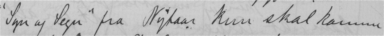"Syn og Segn" fra Nytaar kun skal komme
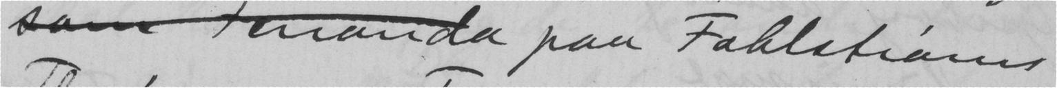paa Fahlstrøms
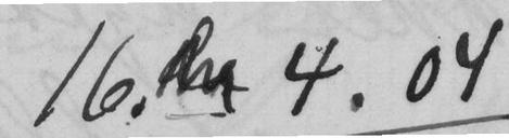16. .4.04
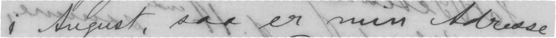i August, saa er min Adresse
